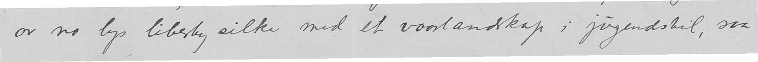av <no> lys liberty silke med et vaarlandskap i jugendstil, saa
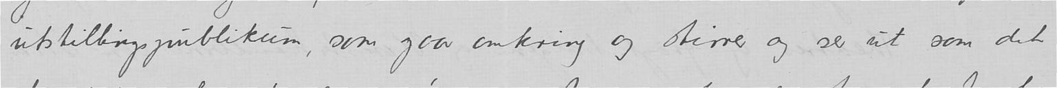utstillingspublikum, som gaar omkring og stirrer og ser ut som
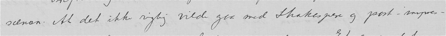scenen. At det ikke rigtig vilde gaa med Shakespere og post-impre-
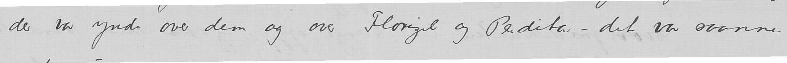der var ynde over dem og over Florizel og Perdita – det var saanne
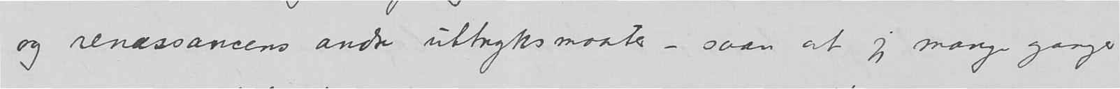og renæssancens andre uttrygsmaater – saan at jeg mange ganger
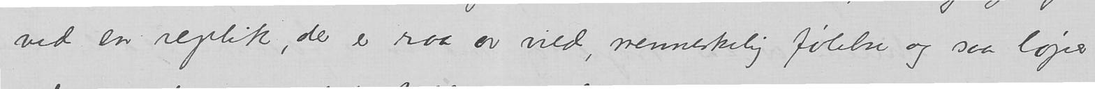ved en replik, der er raa av vild, menneskelig følelse og saa <løper>
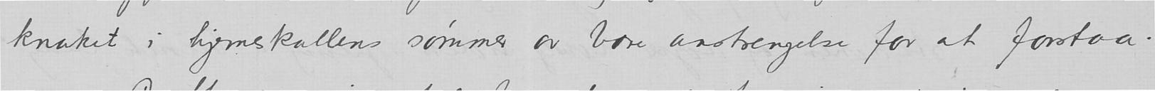det knaket i hjerneskallens sømmer av bare anstrengelse for at forstaa.
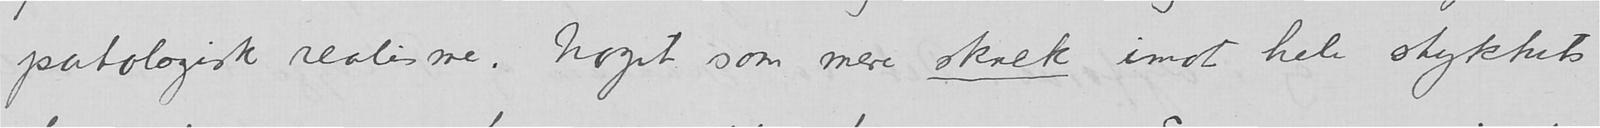patologisk realisme. Noget som mere skrek imot hele stykkets
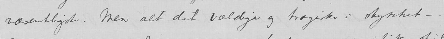væsentligste. Men alt det vældige og tragiske i stykket –.
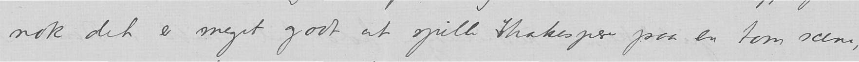nok det er meget godt at spille Shakespere paa en tom scene,
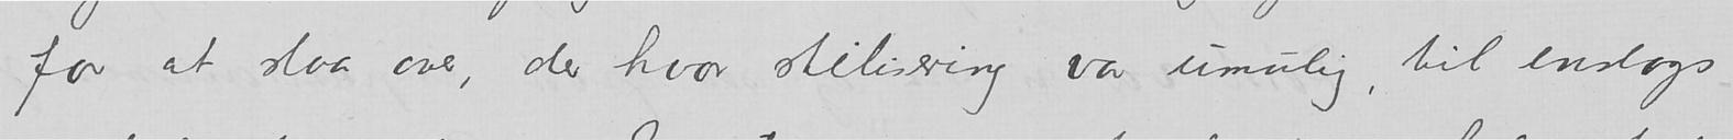for at sltaa over, der hvor stilisering var umulig, til enslags
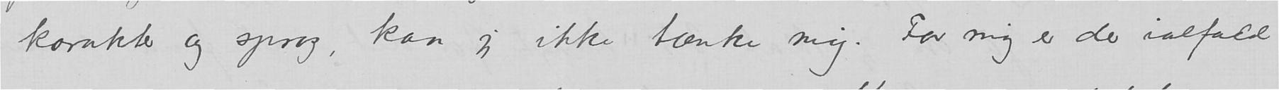karakter og sprog, kan jeg ikke tænke mig. For mig er der ialfald
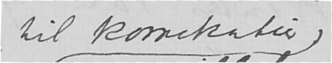til karrekatur
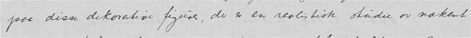paa disse dekorative figurer, der er en realistisk studie av nakent
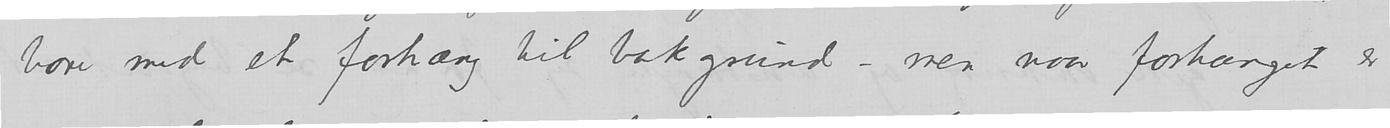bare med et forhæng til bakgrund – men naar forhænget er
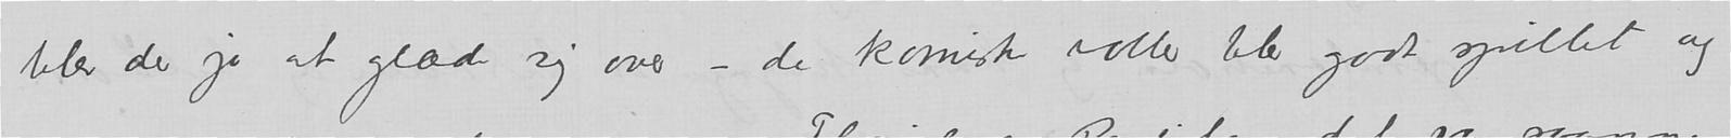blev der jo at glæde sig over – de komiske roller blev godt spillet og
